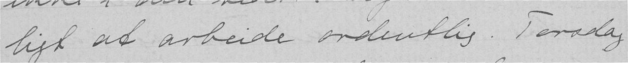ligt at arbeide ordentlig. Torsdag
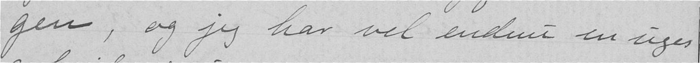gen, og jeg har vel endnu en uges
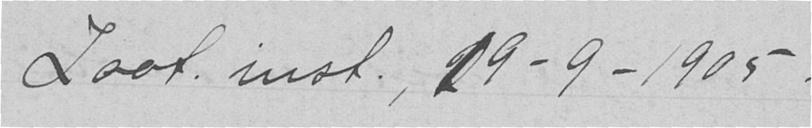Zoot. inst., 29 -9-1905.
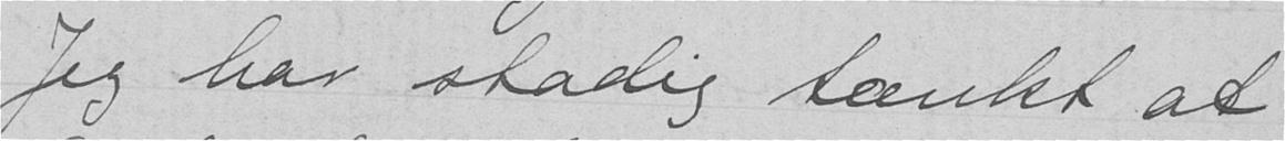Jeg har stadig tænkt at
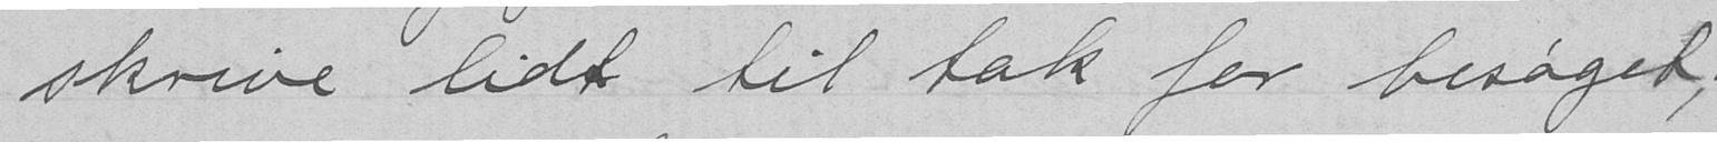skrive lidt til tak for besøget;
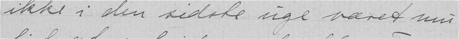ikke i den sidste uge været mu-
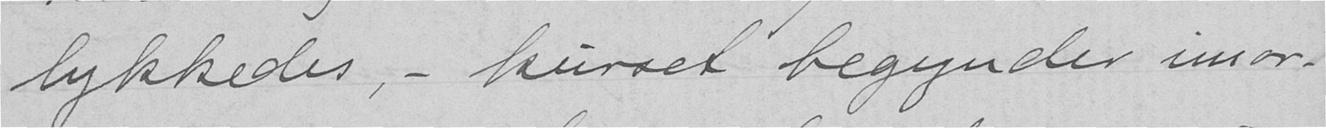lykkedes, - kurset begynder imor-
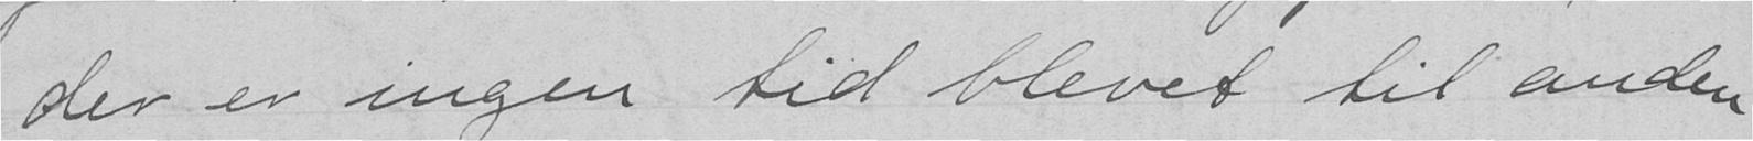der er ingen tid blevet til anden
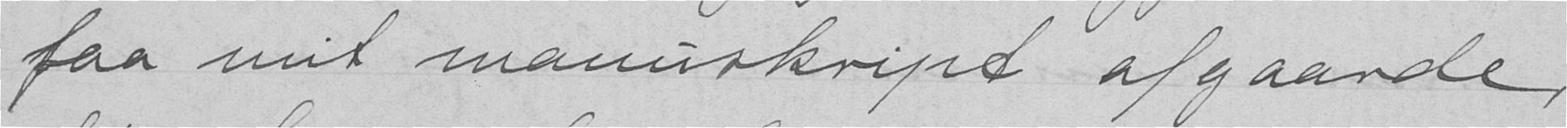faa mit manuskript afgaarde,
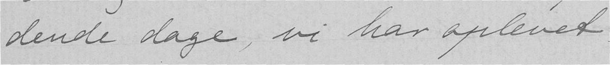dende dage, vi har oplevet;
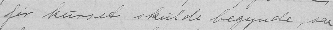før kurset skulde begynde, saa
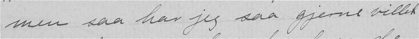men saa har jeg saa gjerne villet
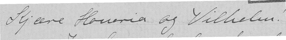Kjære Honoria og Vilhelm!
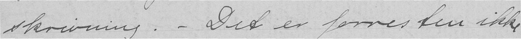skrivning. - Det er forresten ikke
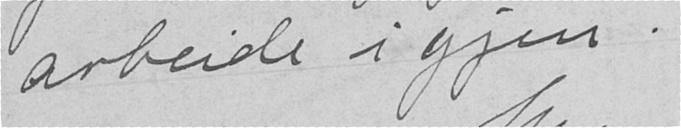arbeide igjen.
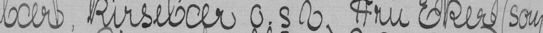bær, kirsebær o.s.v. Fru Eker som
